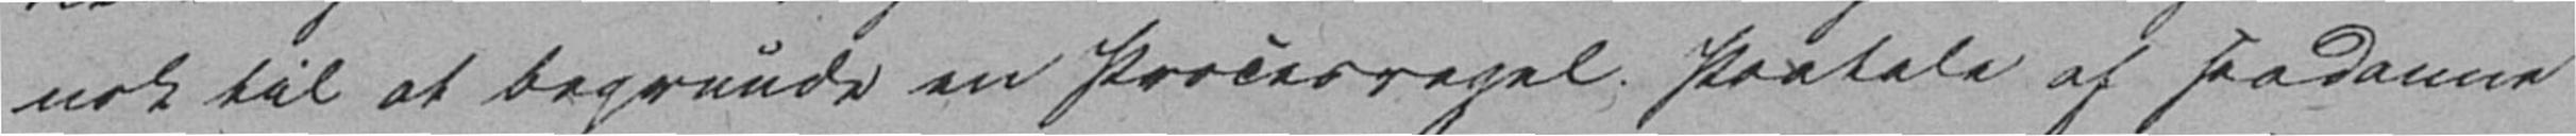nok til at begrunde en Procesregel. Paatale af saadanne
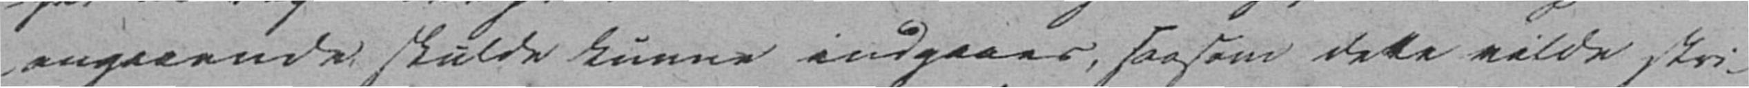angaaende skulde kunne indgaaes, saasom dette vilde skri-
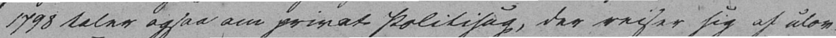1798 taler ogsaa om privat Politisag, der reiser sig af ulov-
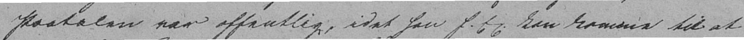Paatalen var offentlig, idet han f. Ex. kan komme til at
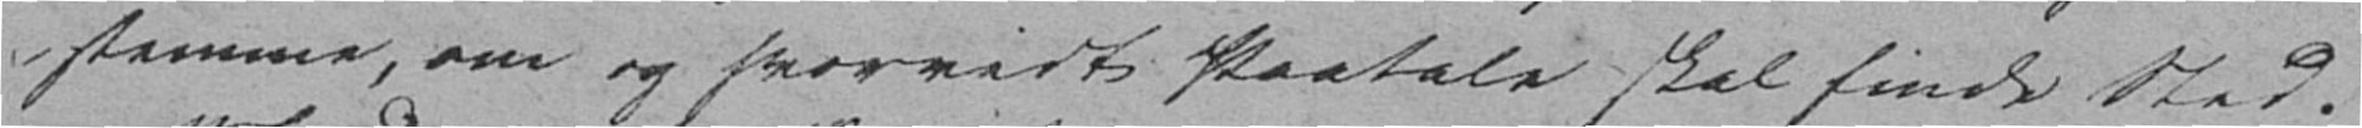stemme, om og hvorvidt Paatale skal finde Sted.
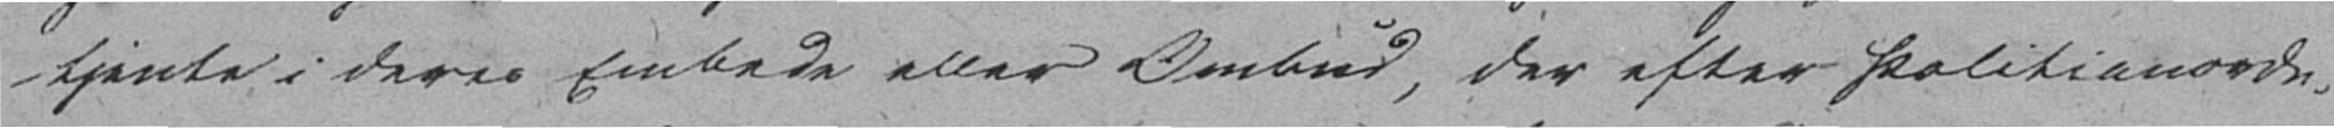tjente i deres Embede eller Ombud, der efter Politianordn,
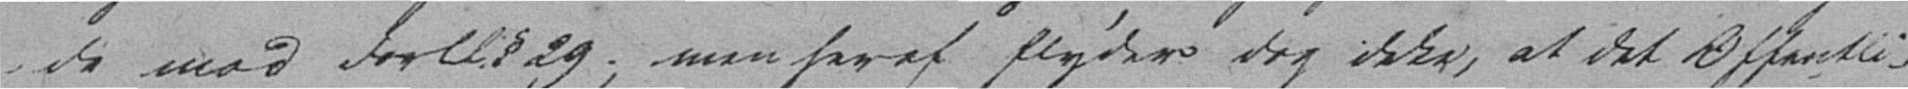de mod Forll. § 29; men heraf flyder dog ikke, at det offentli-
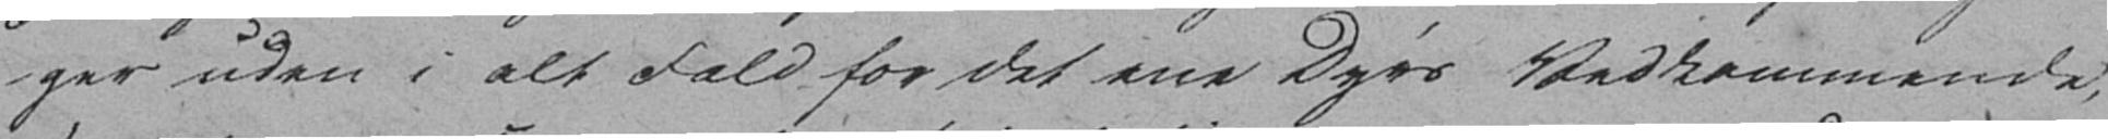ger uden i alt Fald for det ene Dyrs Vedkommende,
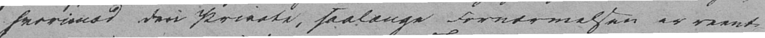hvorimod den Private, saalænge Fornærmelsen er reent
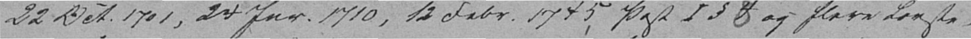22 Oct. 1701, 24 Jnr. 1710, 12 Febr. 1745, Post I § 4 og flere Lovste-
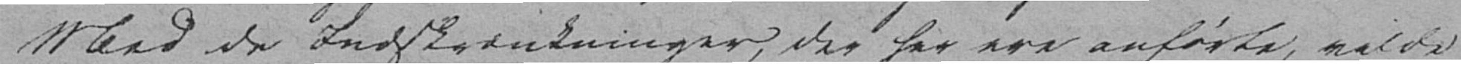Med de Indskrænkninger, der her ere anførte, vilde
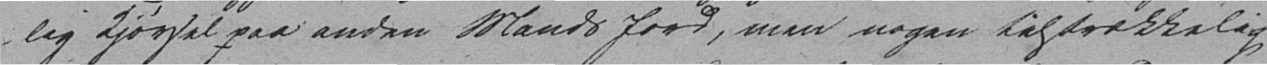lig Kjørsel paa anden Mands Jord, men nogen tilstrækkelig
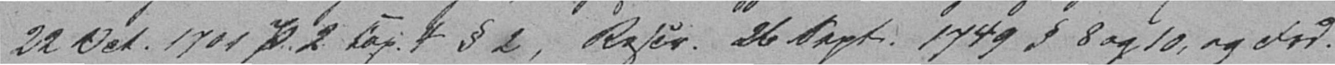22 Oct. 1701 P. 2 Cap. 4 § 2, Rescr. 26 Sept. 1749 § 8 og 10, og Frd.
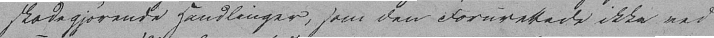skadegjørende Handlinger, som den Forurettede ikke ved
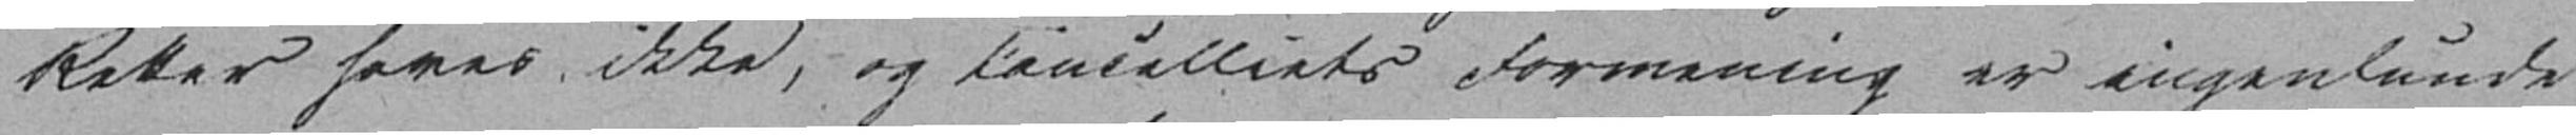Retter haves ikke, og Cancelliets Formening er ingenlunde
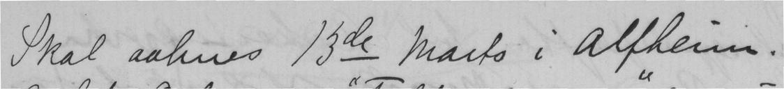Skal aabnes 13de Marts i Alfheim.
